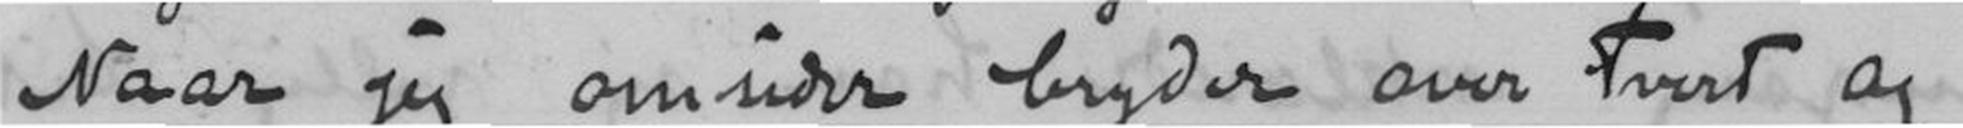Naar jeg omsider bryder over tvert og
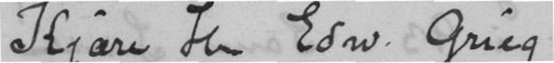Kjære Hr. Edw. Grieg
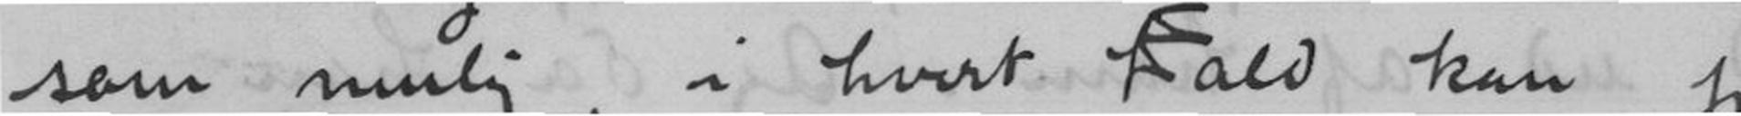som mulig, i hvert Fald kan jeg
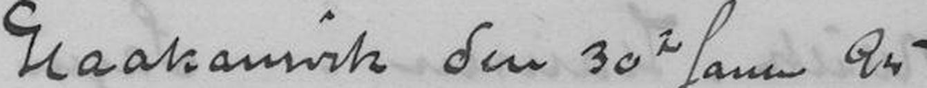Haakonsvik den 30t Januar 95
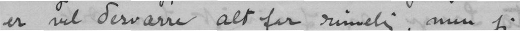er vel Desværre altfor rimelig, men jeg
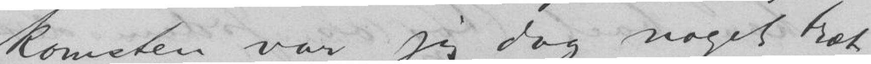komsten var jeg dog noget træt
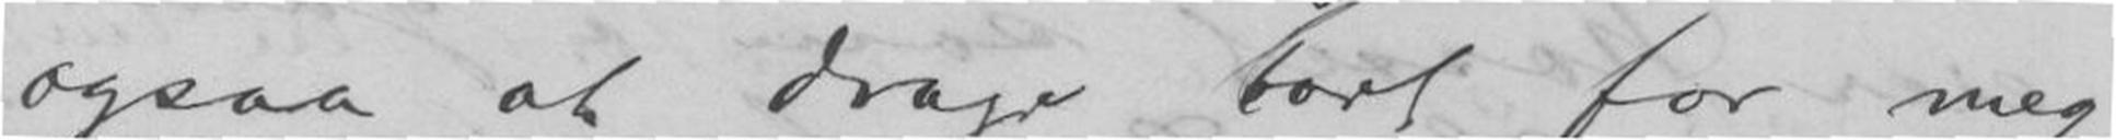ogsaa at drage bort for meg
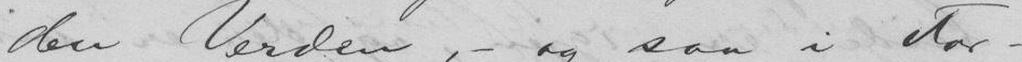den Verden, - saa i For-
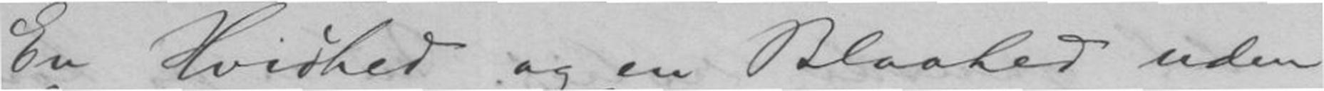En Hvidhed og en Blaahed uden
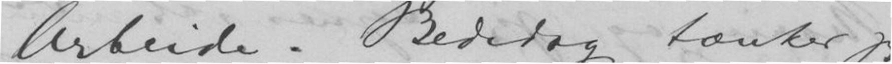Arbeide. Bededag tænker jeg
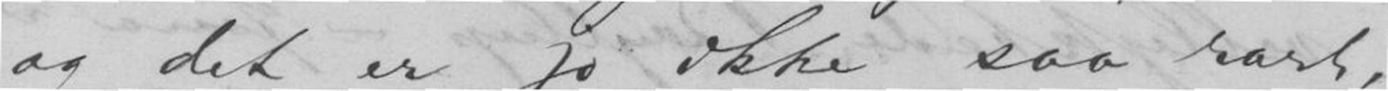og det er jo ikke saa rart,
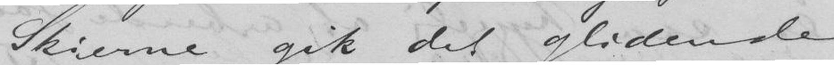Skierne gik det glidende
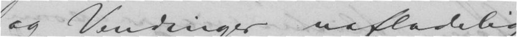og Vendinger uafladelig.
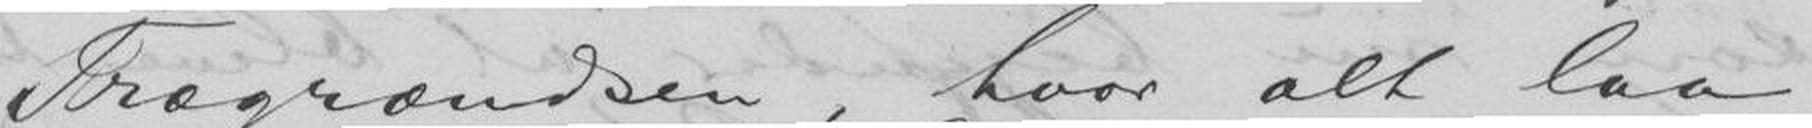Trægrændsen, hvor alt laa
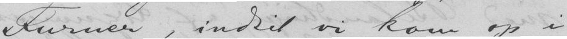Furuer, indtil vi kom op i
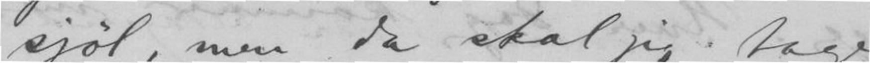sjøl, men da skal jeg tage
